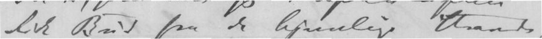Fik Bud fra de hjemlige Strand,
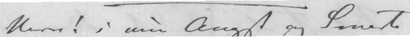Herre! i min Angst og Smerte
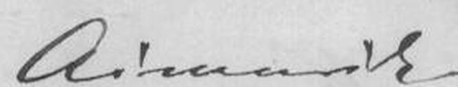Aimerik
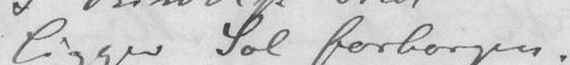Ligger Sol forborgen.
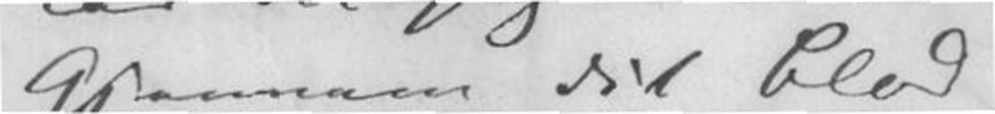gjennem dit Blod -
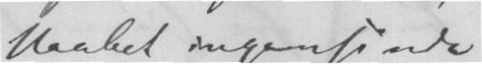Haabet ingensinde
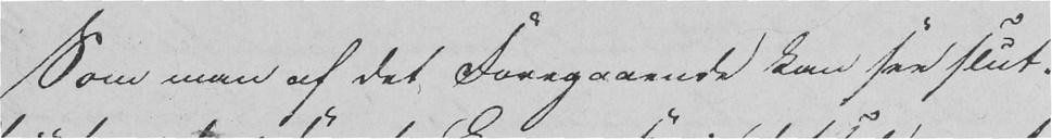Som man af det Foregaaende kan see slut-
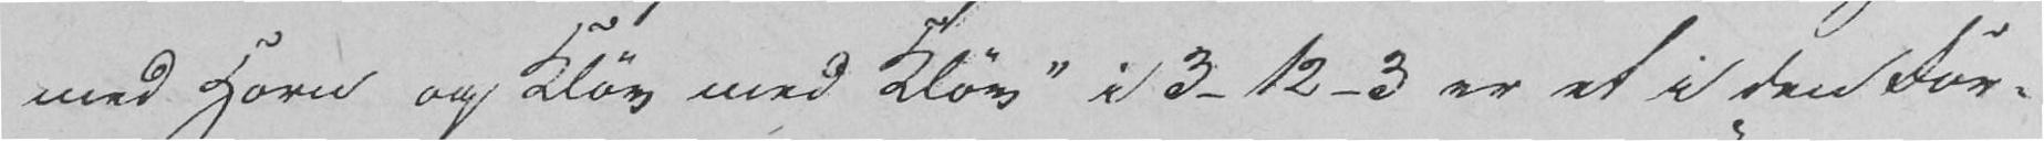med Horn og Kløv med Kløv" i 3-12-3 er et i den For-
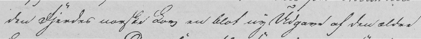den Fjerdes norske Lov en blot ny Udgave af den ældre
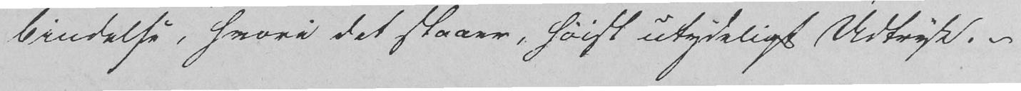bindelse, hvori det staaer, høist utydeligt Udtryk. -
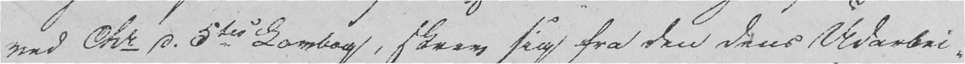ved Chr d. 5tes Lovbog, skrev sig fra den dens Udarbei-
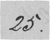25.
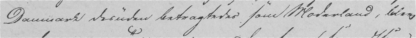Danmark desuden betragtedes som Moderland, blev
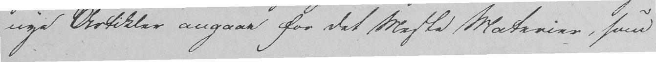nye Artikler angaar for det Meste Materier, som
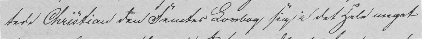tede Christian den Femtes Lovbog sig i det Hele meget
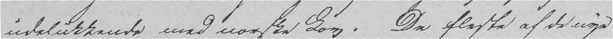udelukkende med norske Lov. De fleste af de nye
